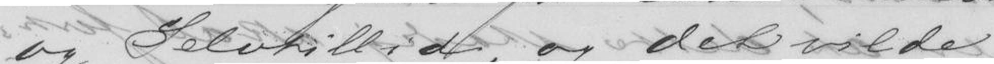og Selvtillid, og det vilde
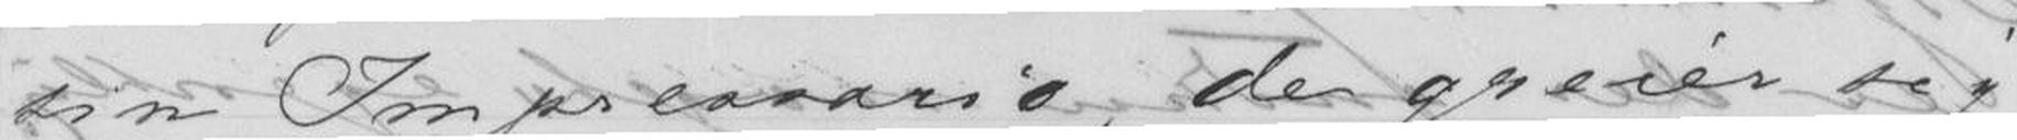sin Impressario, de greier sig
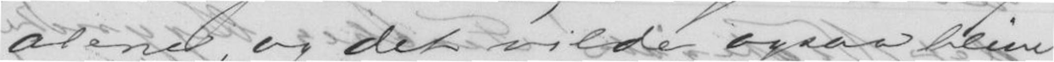alene, og det vilde ogsaa blive
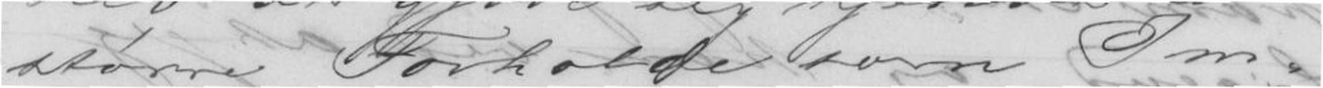større Forholde som Im-
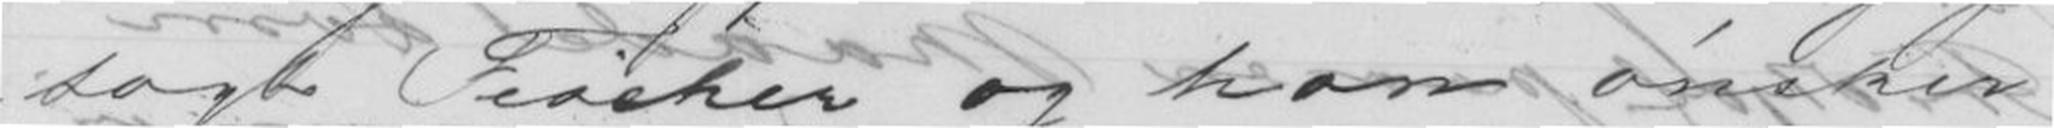sagt Fischer og han ønsker
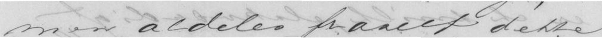men aldeles fraseet dette
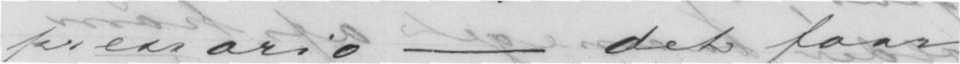pressario- det faar
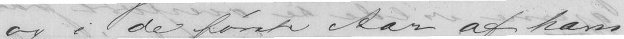og i de første Aar af hans
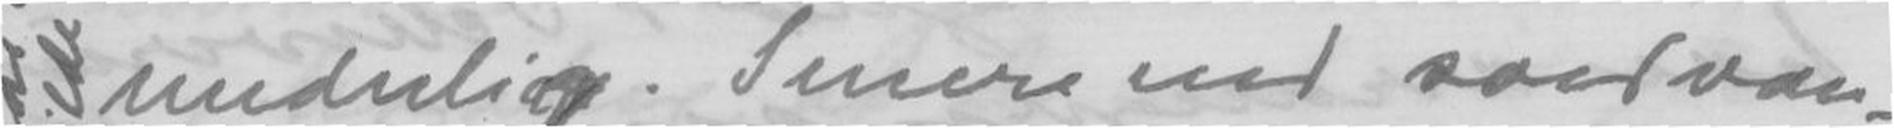underlig. Senere end saed van-
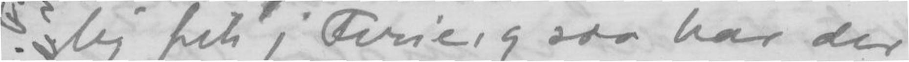lig fik j Ferie og saa har der
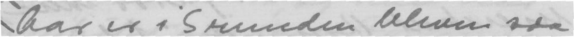Aar er i Grunden bleven saa
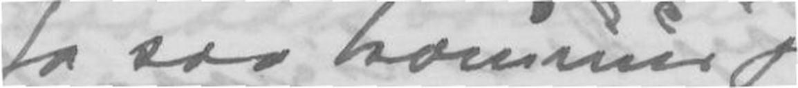Ja saa kommer jeg
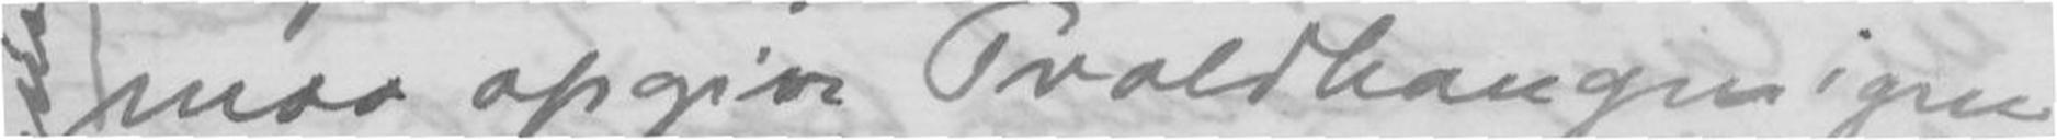maa opgive Troldhaugen igen
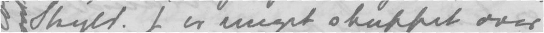Skyld. J er meget skuffet over
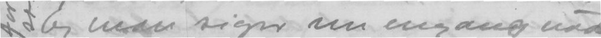Og man siger nu engang nok
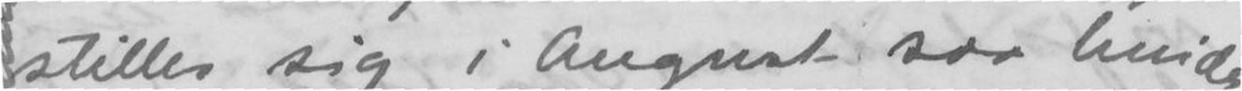stiller sig i August saa hindren-
